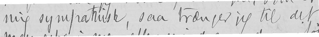mig sympathisk, saa trænger jeg til det
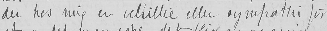der hos mig er velvillie eller sympathi for
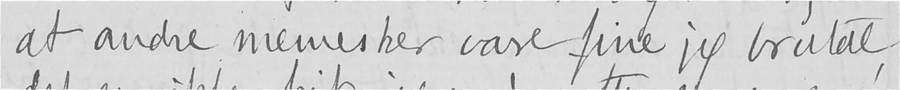at andre mennesker vare fine jeg brutal,
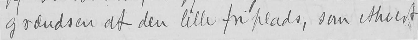grændsen af den lille friplads, som ethvert
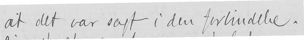at det var sagt i den forbindelse.
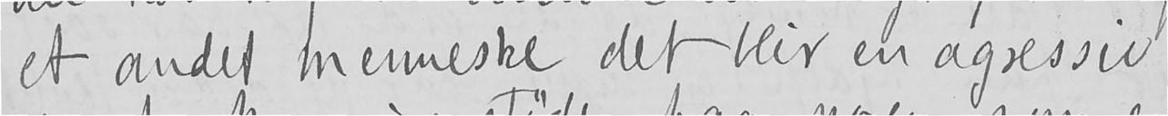et andet menneske, det blir en agressiv
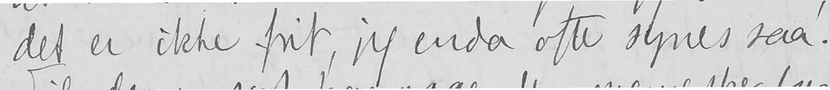det er ikke frit, jeg enda ofte synes saa.
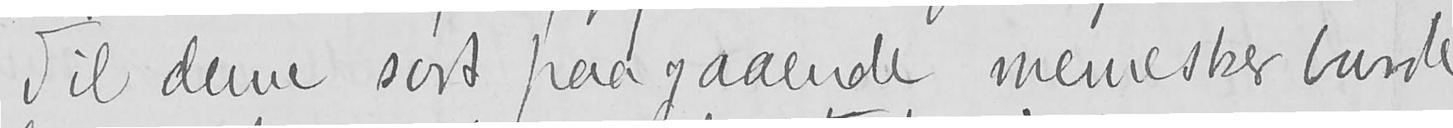Til denne sort paagaaende mennesker burde
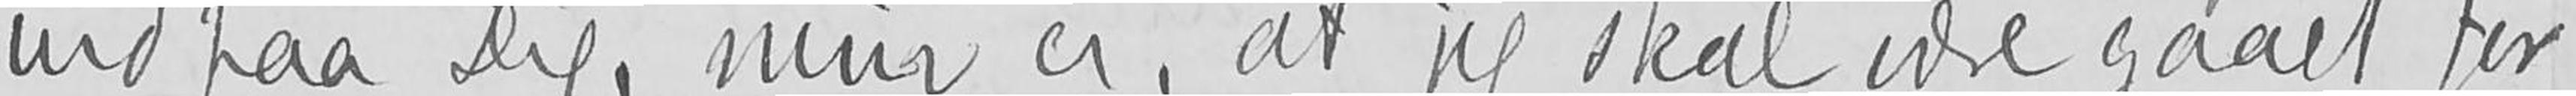ind paa Dig, min er, at jeg skal være gaaet for
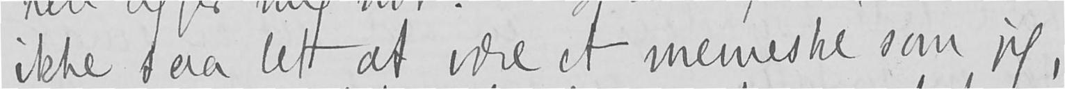ikke saa lett at være et menneske, som jeg,
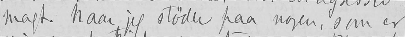magt. Naar jeg støder paa nogen, som er
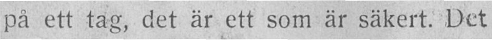på ett tag, det är ett som är säkert. Det
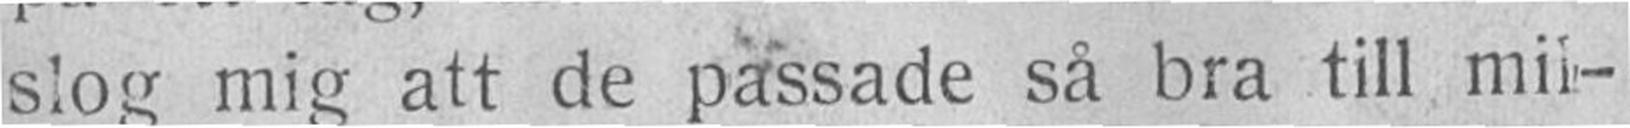slog mig att de passade så bra till mil-
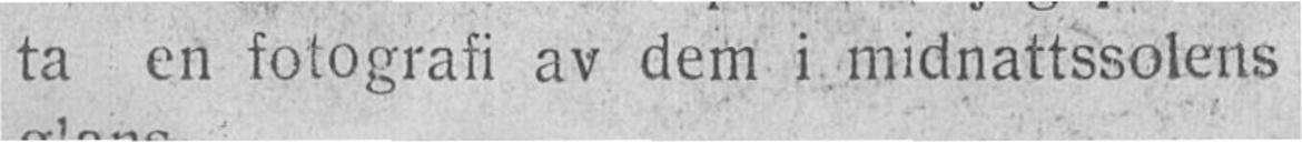ta en fotografi av dem i midnattssolens
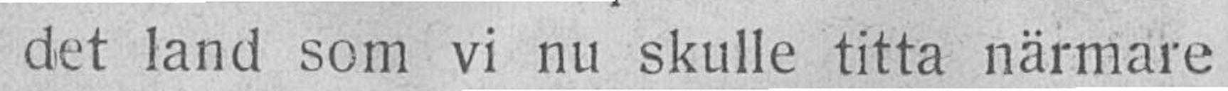det land som vi nu skulle titta närmare
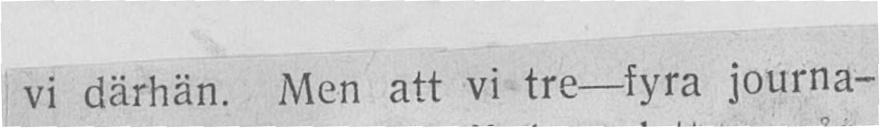vi därhän. Men att vi tre-fyra journa-
